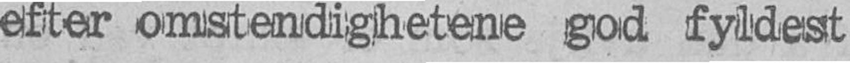efter omstendighetene god fyldest
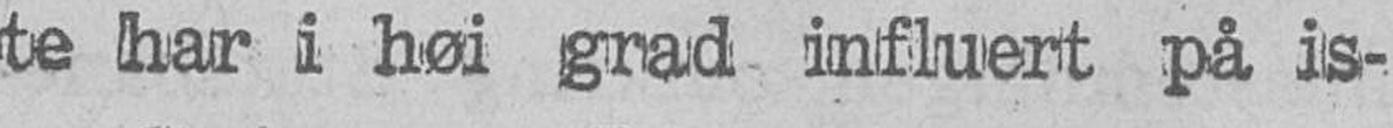te har i høi grad influert på is-
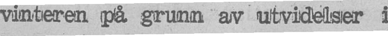vinteren på grunn av utvidelser i
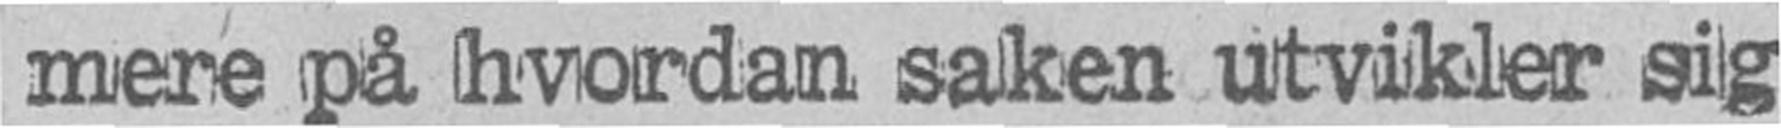mere på hvordan saken utvikler sig.
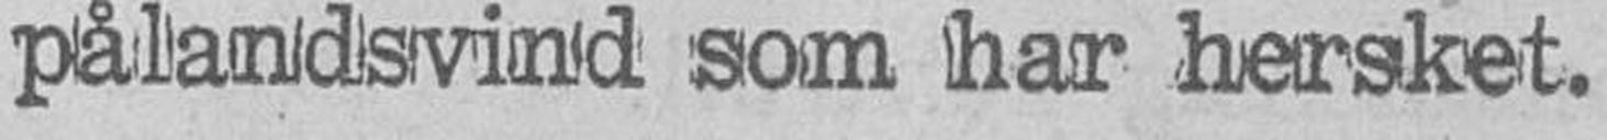pålandsvind som har hersket.
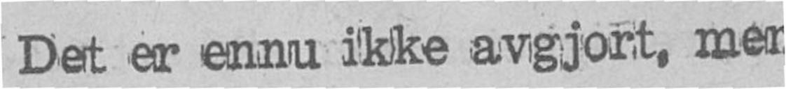- Det er ennu ikke avgjort, men
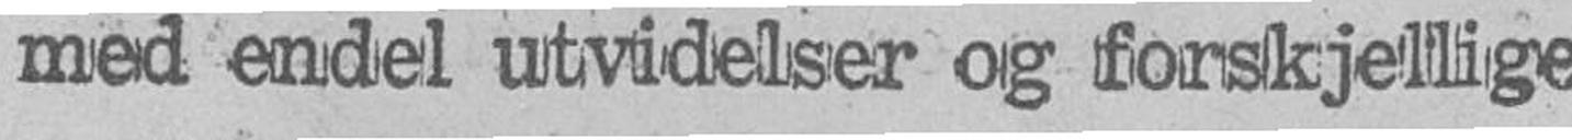med endel utvidelser og forskjellige
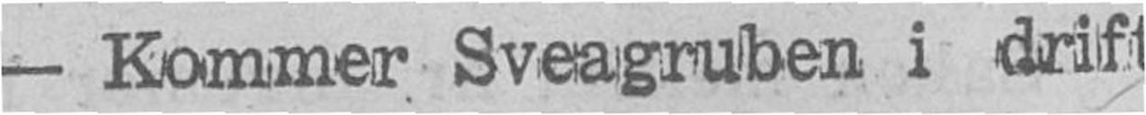- Kommer Sveagruben i drift
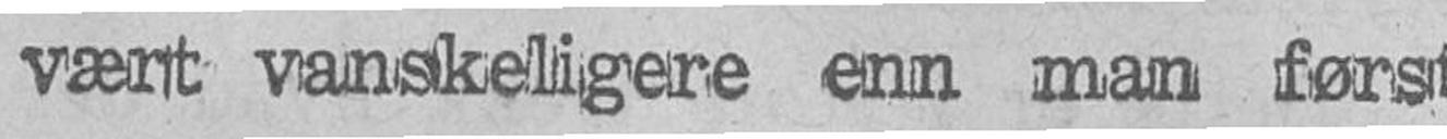vært vanskeligere enn man først
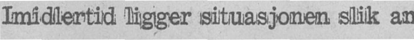Imidlertid ligger situasjonen slik an
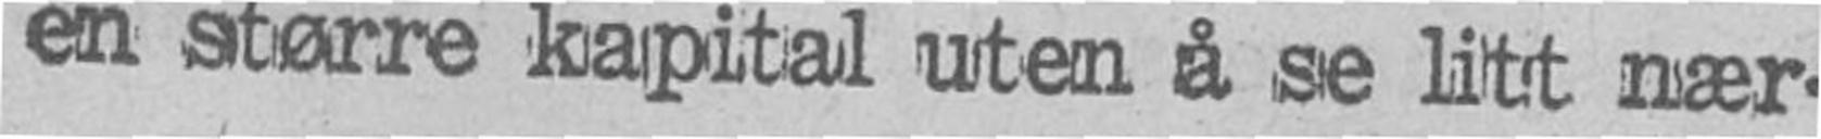en større kapital uten å se litt nær-
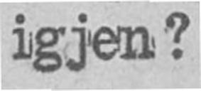igjen?
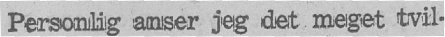Personlig anser jeg det meget tvil-
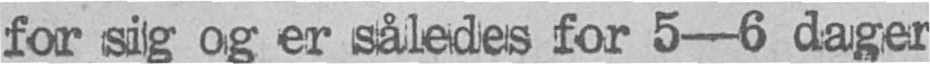for sig og er således for 5 - 6 dager
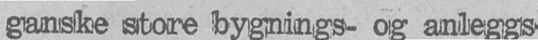ganske store bygnings- og anleggs-
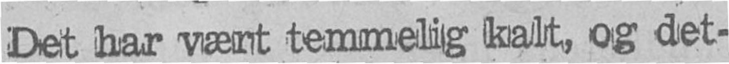Det har vært temmelig kalt, og det-
